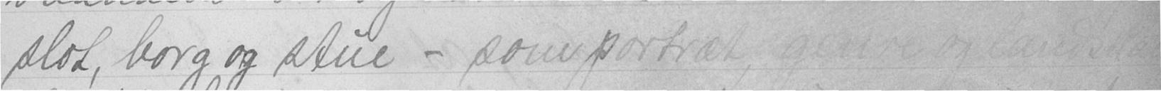slot, borg og stue - som portræt, genre og landskab,
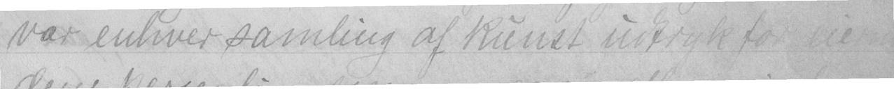var enhver samling af kunst udtryk for eier-
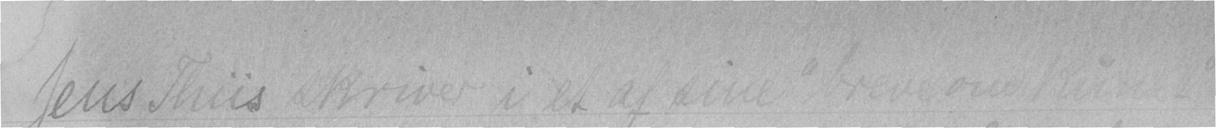Jens Thiis skriver i et af sine "breve om kunst" i
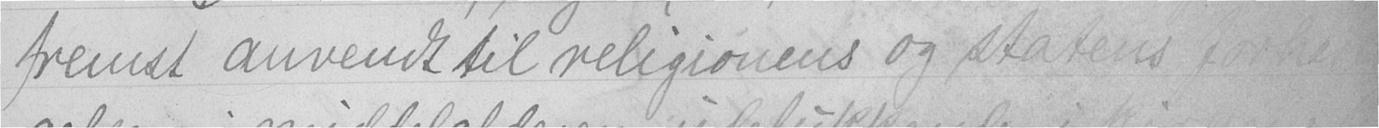fremst anvendt til religionens og statens forherli-
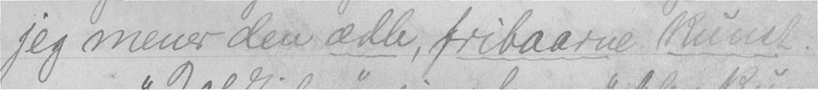jeg mener den ædle, fribaarne kunst.
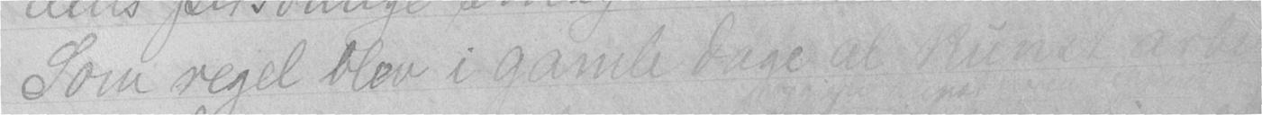Som regel blev i gamle dage al kunst arbeidet
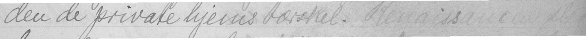den de private hjems tærskel. Renaissancen slod
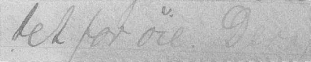tet for øie. Der
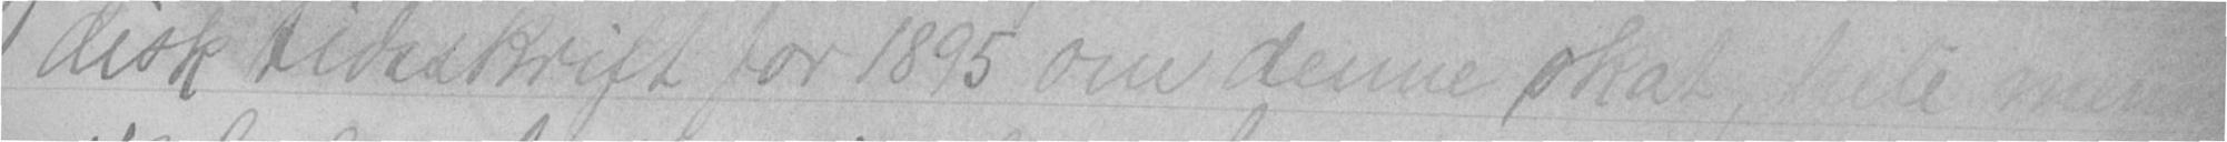disk tidsskrift for 1895 om denne skat, hele menne-
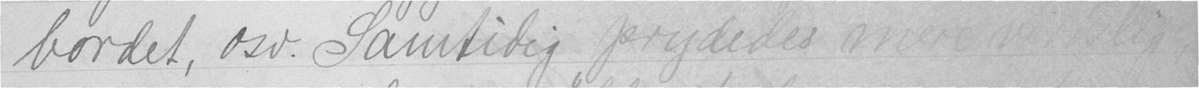bordet, osv. Samtidig prydedes mere verdslig,
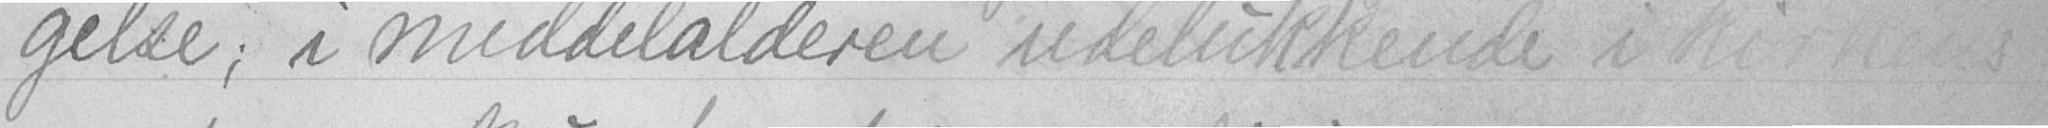gelse; i middelalderen udelukkende i kirkens
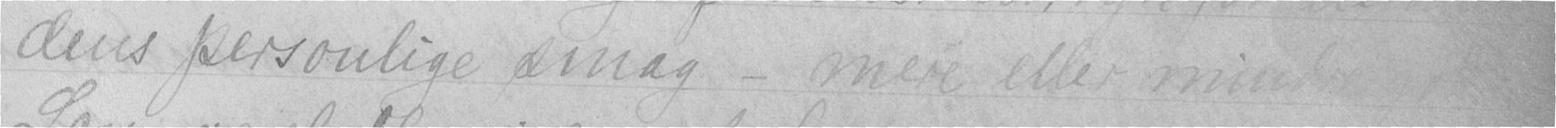dens personlige smag - mere eller mindre godt
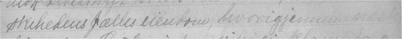skehedens fælles eiendom, hvorigjennem næste
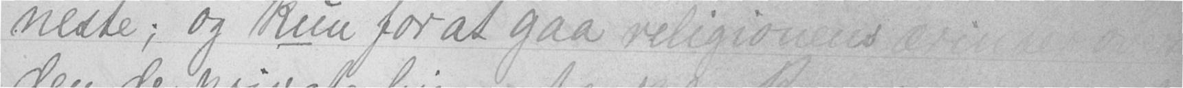neste; og kun for at gaa religionens ærinder over
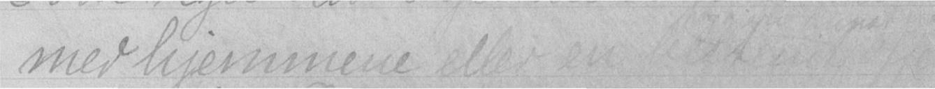med hjemmene eller en bestemt
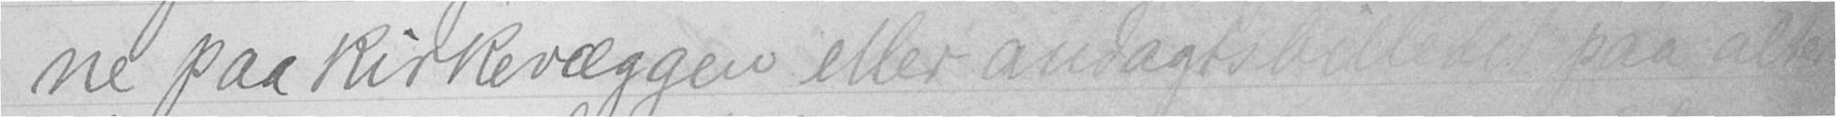ne paa kirkevæggen eller andagtsbilledet paa alter-
